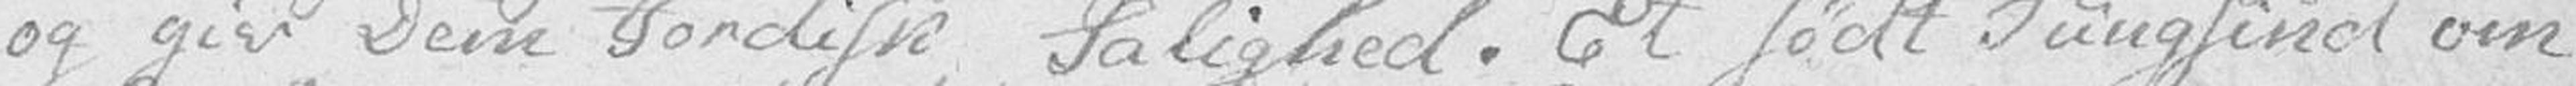og giv Dem Jordisk Salighed. Et sødt Tungsind om
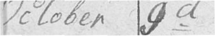October 9d
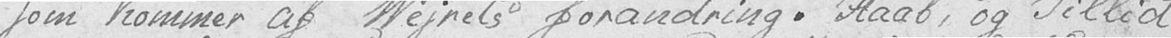som kommer af Vejrets forandring. Haab, og Tillid
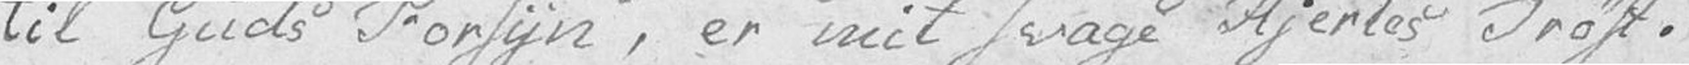til Guds Forsyn, er mit svage Hjertes Trøst. –
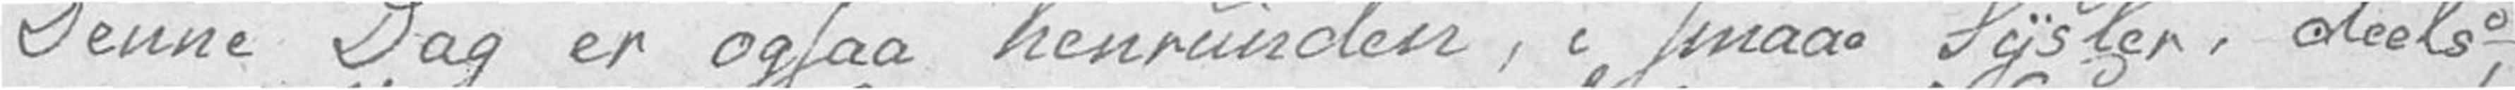Denne Dag er ogsaa henrunden, i smaae Sysler, deels
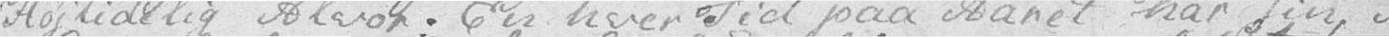Højtidelig Alvor. En hver Tid paa Aaret har sin
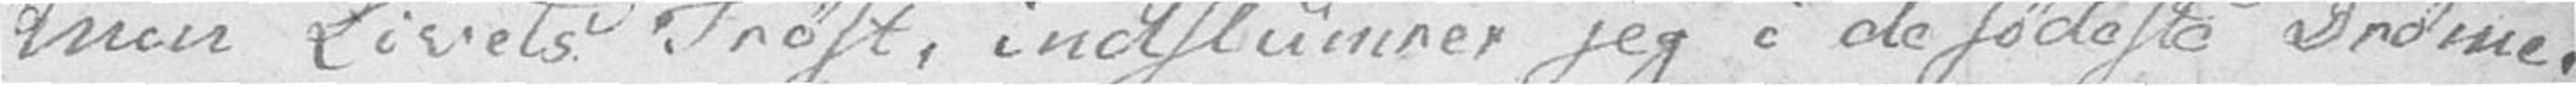min Livets Trøst, indslumrer jeg i de sødeste Drøme.
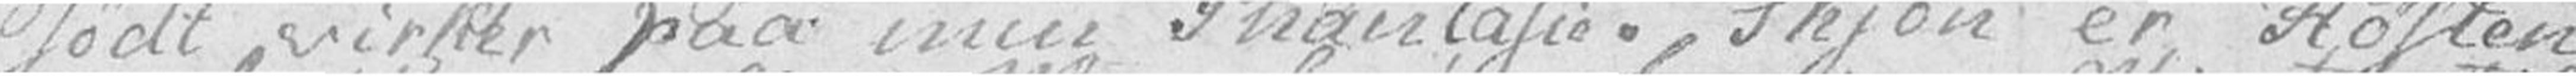sødt virker paa min Phantasie. Skjøn er Høsten
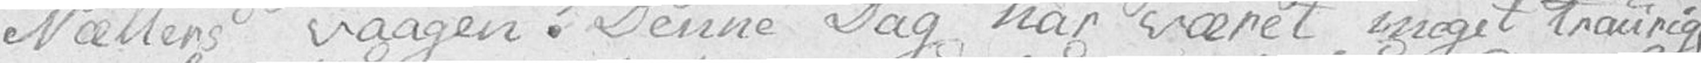Nætters vaagen. Denne Dag har været meget traurig,
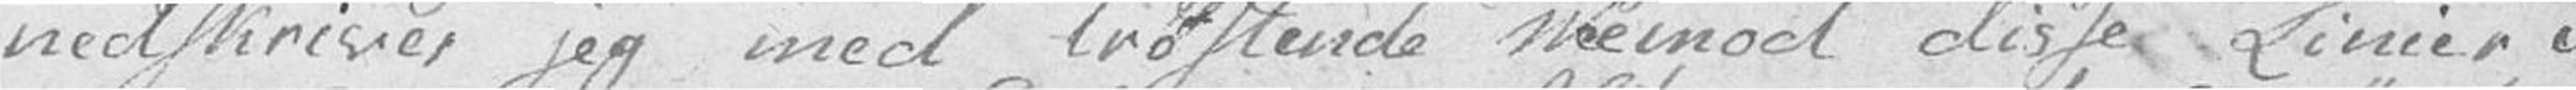nedskriver jeg med trøstende Veemod disse Linier i
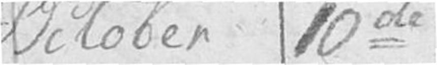October 10de
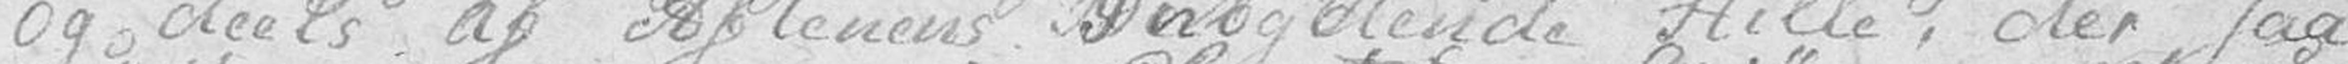og deels af Aftenens Inbydende Stille, der saa
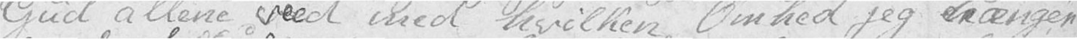Gud allene veed med hvilken Ømhed jeg trænger
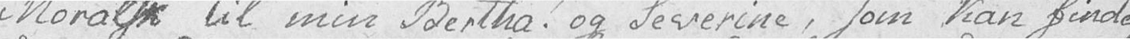Moralsk til min Bertha! og Severine, som kan finde
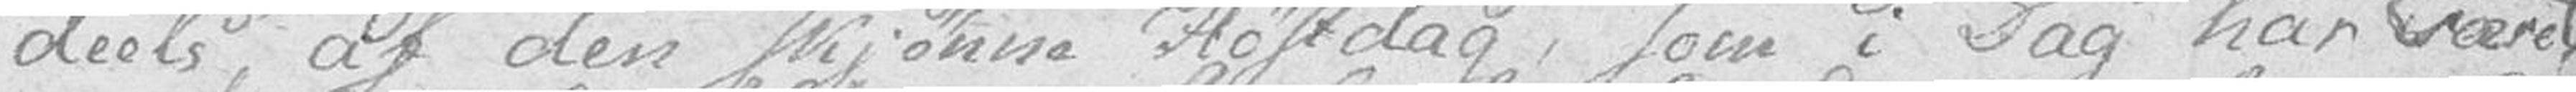deels af den skjønne Høstdag, som i Dag har været,
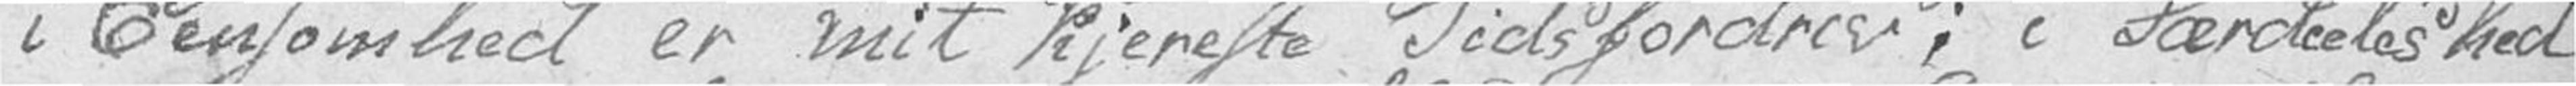i Eensomhed er mit Kjereste Tidsfordriv; i Særdeeleshed
